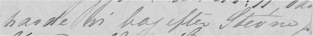havde vi bag efter Stevne fra
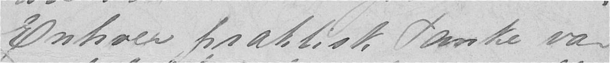Enhver praktisk Tanke var
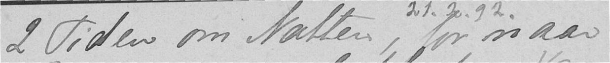2 Tiden om Natten, for naar
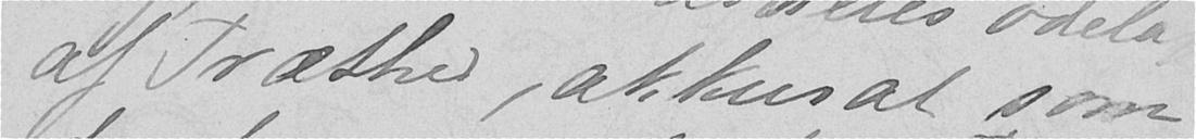af Træthed, akkurat som-
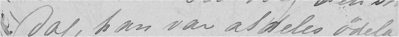Dag, han var aldeles ødelagt
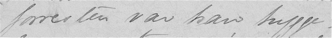forresten var han hygge-
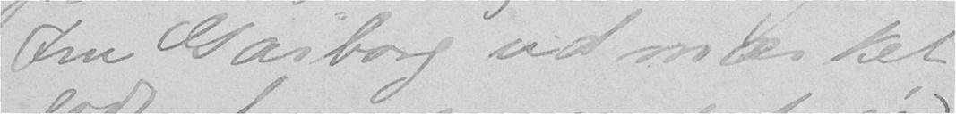Fru Garborg udmærket
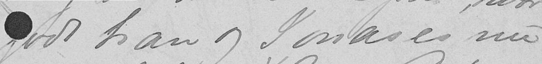godt han og Jonases nu
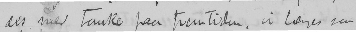det med tanke paa fremtiden, vi længes saa
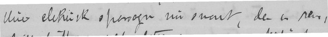blive elektrisk sporvogn nu snart, der er ren,
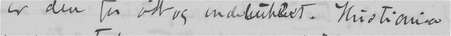er den for ødt og indelukket. Kristiania
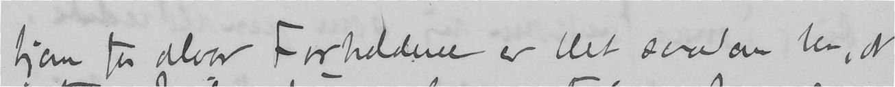hjem for alvor Forholdene er blet saadan her, at
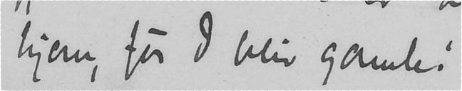hjem, før I blir gamle!
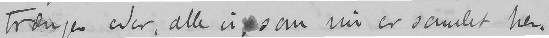trænger eder, alle vi, som nu er samlet her
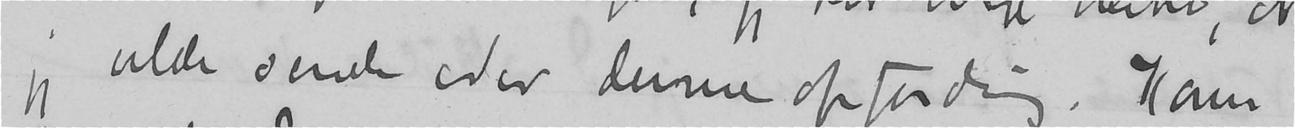jeg vilde sende eder denne opfording. Kom
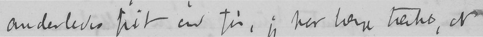anderledes frit end før, jeg har længe tænkt, at
